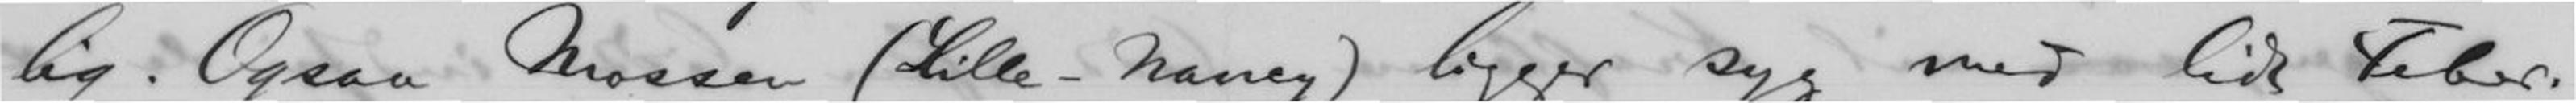lig. Ogsaa Mossen (Lille-Nancy) ligger syg med lidt Feber.
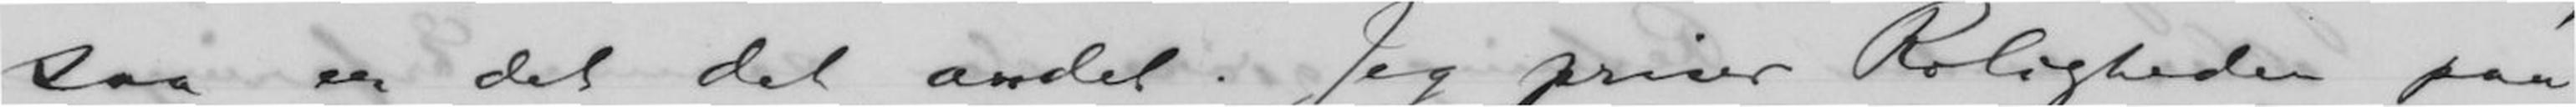saa er det det andet. Jeg priser Roligheden paa
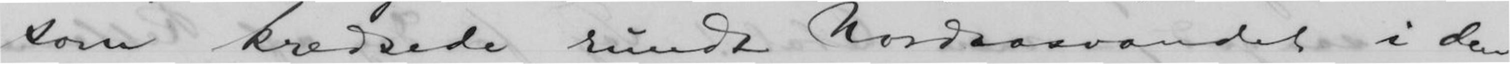som kredsede rundt Nordaasvandet i den
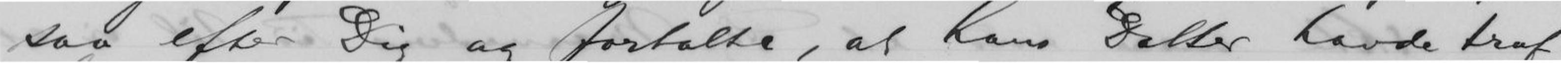saa efter Dig og fortalte, at hans Datter havde truf-
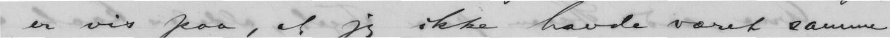er vis paa, at jeg ikke havde været samme
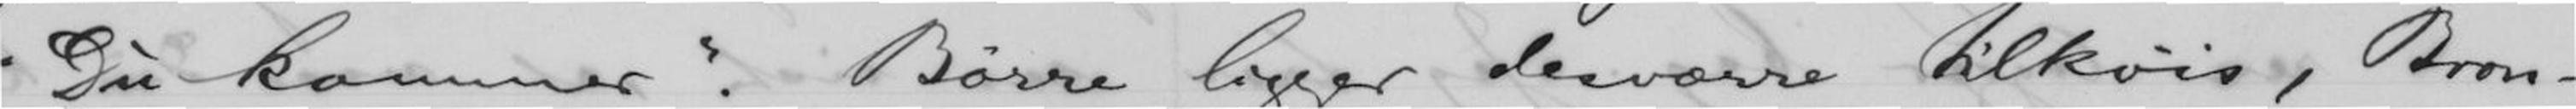Du kommer". Børre ligger desværre tilkøis, Bron-
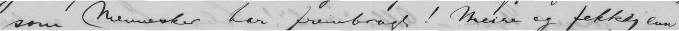som Mennesker har frembragt! Meire eg fekk, enn
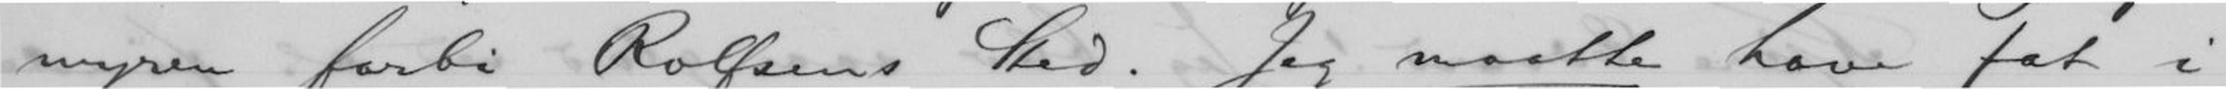myren forbi Rolfsens Sted. Jeg maatte have fat i
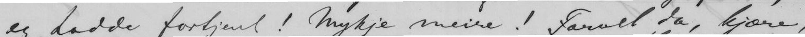eg hadde fortjent! Mykje meire! Farvel da, kjære,
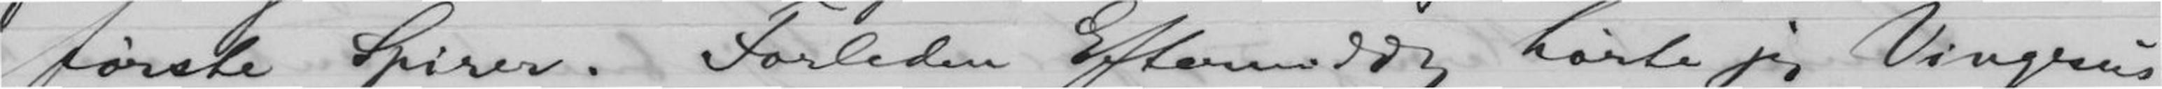første Spirer. Forleden Eftermiddag hørte jeg Vingesus
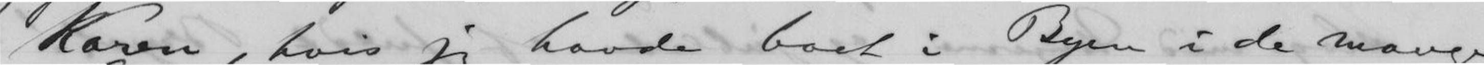Karen, hvis jeg havde boet i Byen i de mange
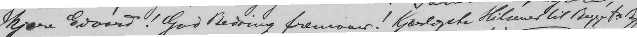kjære Edvard! God Bedring fremover! Kjærligste Hilsener til Begge fra Begge!
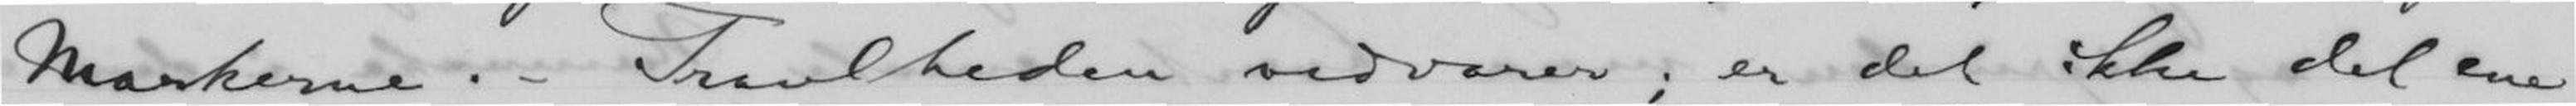Markerne. - Travlheden vedvarer; er det ikke det ene,
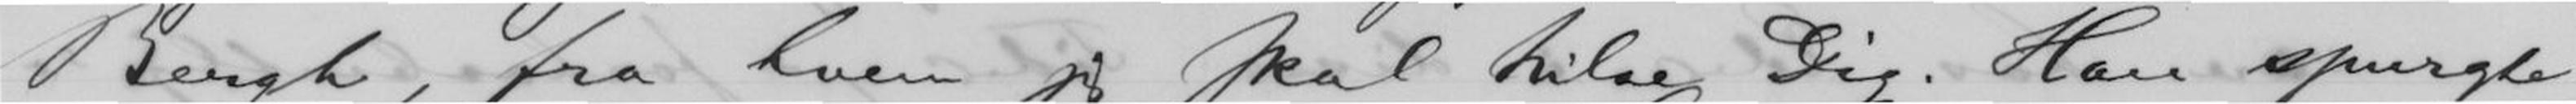Bergh, fra hvem jeg skal hilse Dig. Han spurgte
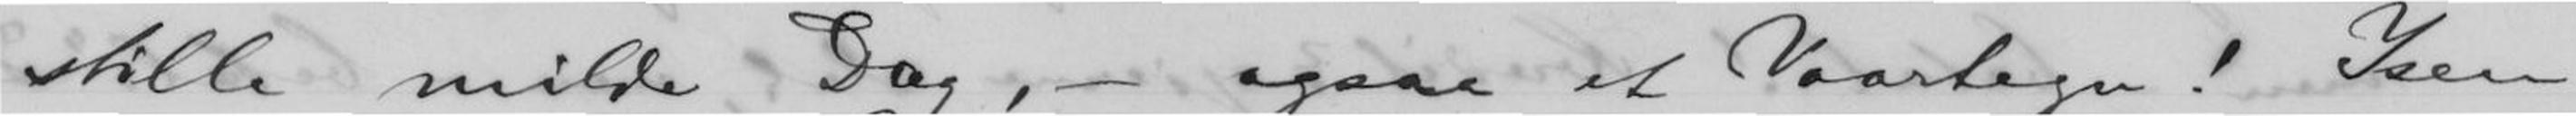stille milde Dag, - ogsaa et Vaartegn! Isen
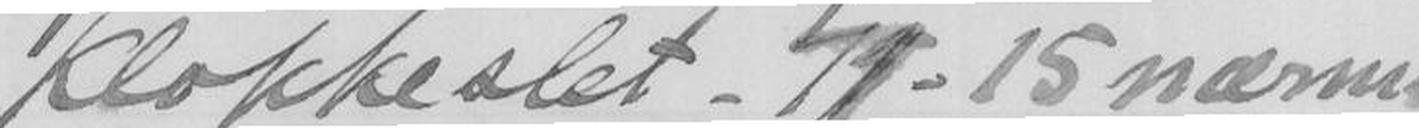klokkeslet - 11.15 nærm
